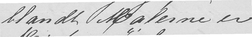blandt Malerne er
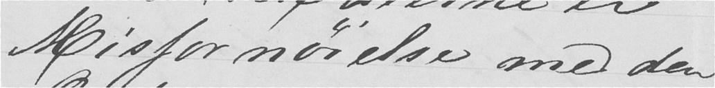Misfornøielse med den
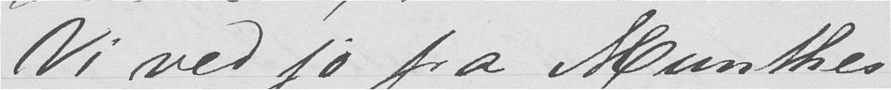Vi ved jo fra Munthes
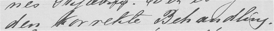den korrekte Behandling.
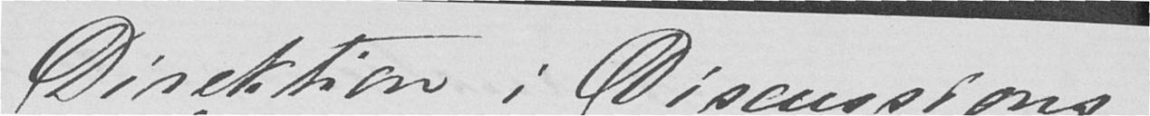Direktion i Discussions-
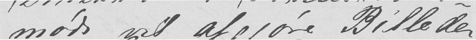møde vil afgjøre Billeder-
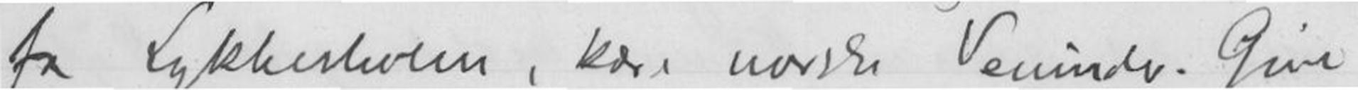fra Lykkeskolen, kære norske Veninder. Give
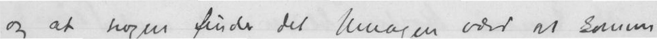og at nogen finder det Umagen værd at komme
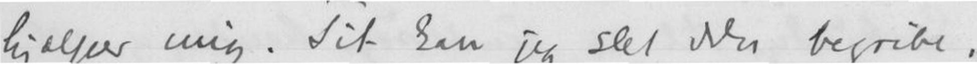hjælper mig. Tit kan jeg slet ikke begribe,
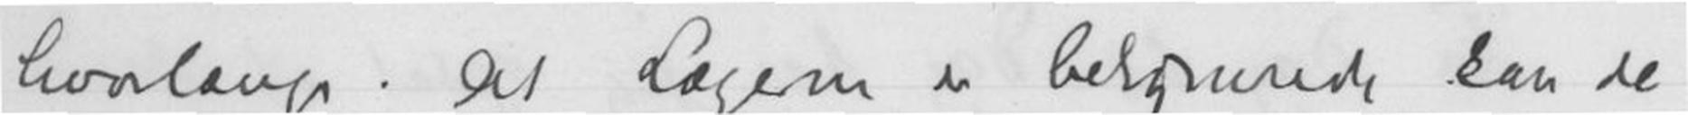hvorlænge. At Lægene er bekymrede kan de
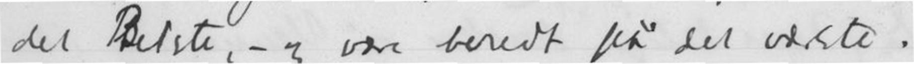det Bedste, - og være beredt på det værste.
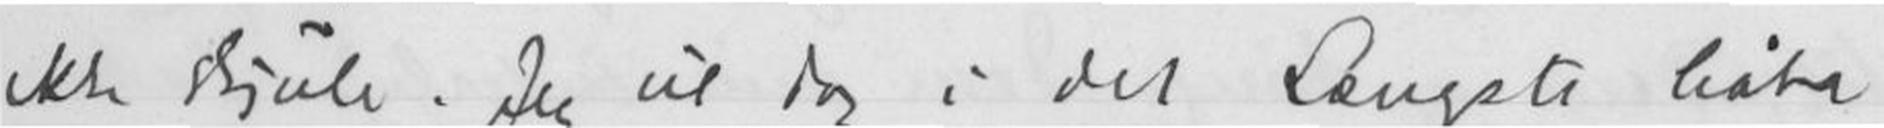ikke skjule. Jeg vil dog i det Længste håbe
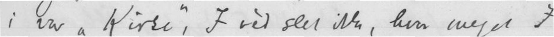i vor Kirke, I véd slet ikke, hvor meget I
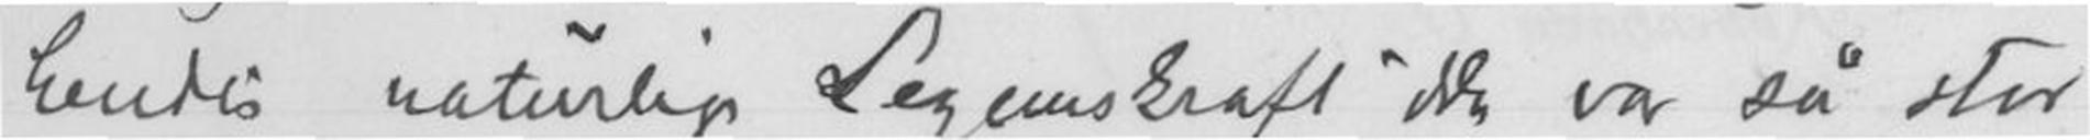hendes naturlige Legemskraft ikke var så stor
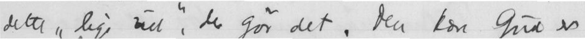dette lige ud, der gør det. Den kære Gud er
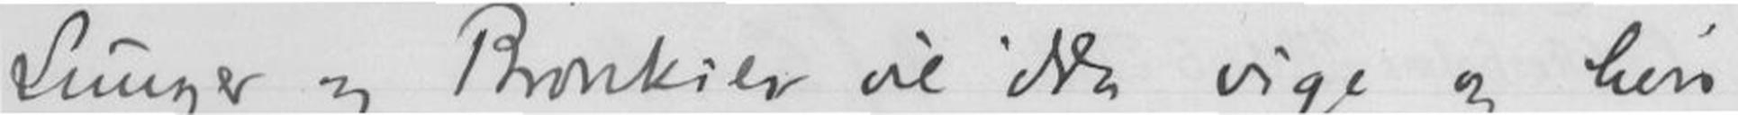Lunger og Bronkier vil ikke vige og heri
